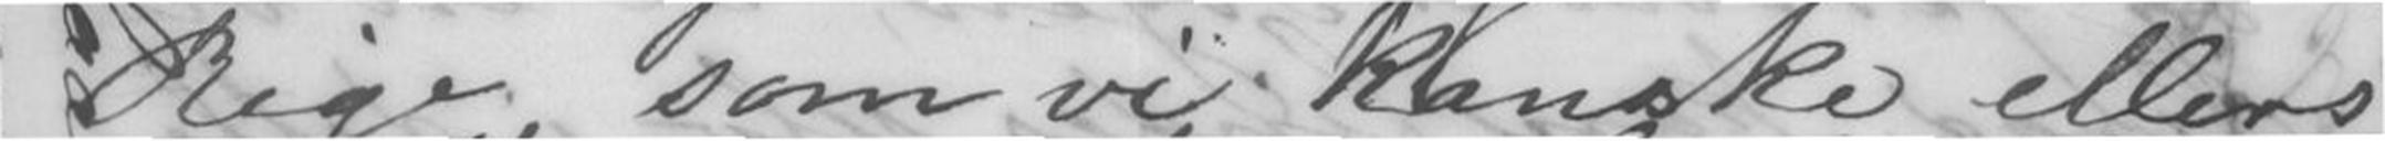Rige, som vi kanske ellers
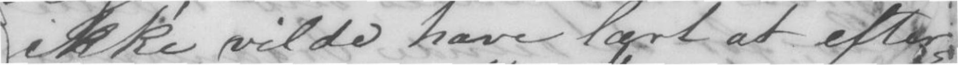ikke vilde have lært at efter-
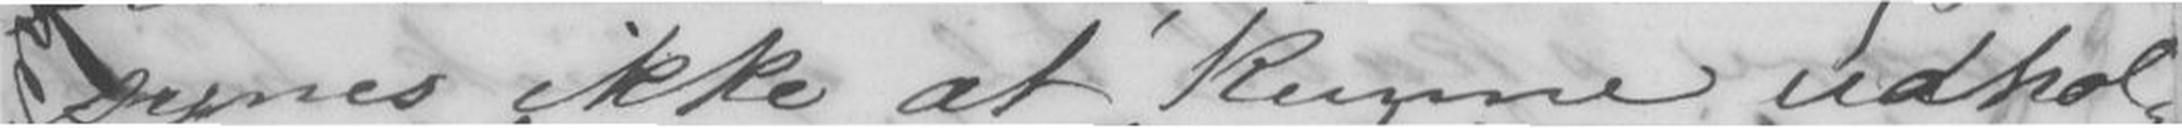synes ikke at kunne udhol-
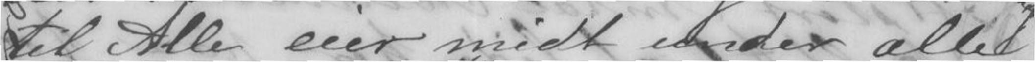til Alle eier midt under alle
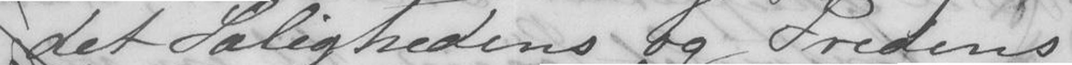det Salighedens og Fredens
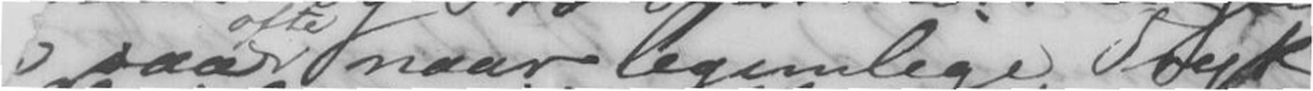saa ofte naar legemlige Tryk
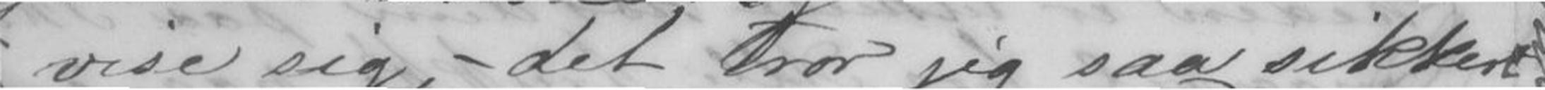vise sig, - det tror jeg saa sikkert
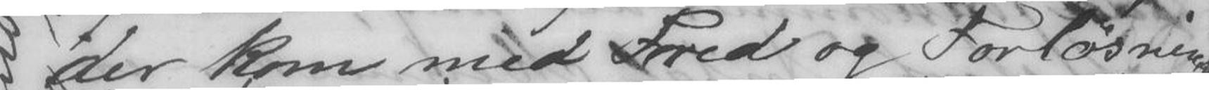der kom med Fred og Forløsning
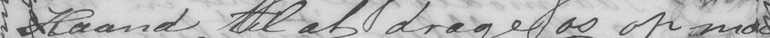Haand til at drage os op mod
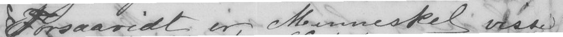Forsaavidt er Mennesket visse-
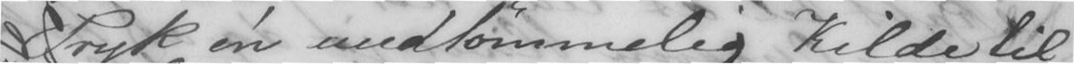Tryk én uudtømmelig Kilde til
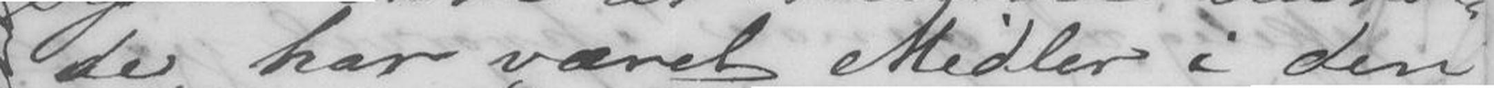de, har været Midler i den
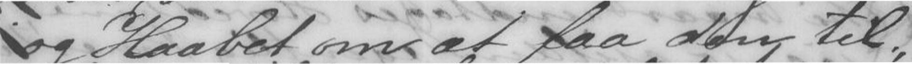og haab om at faa den til-
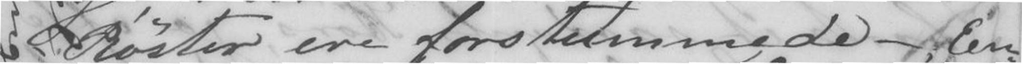Røster ere forstummende - En-
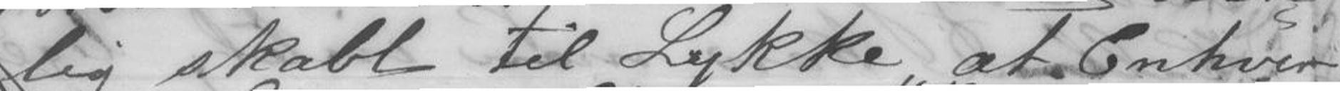lig skabt til Lykke, at Enhver
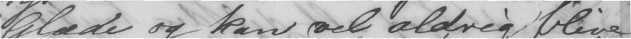Glæde og kan aldrig blive
